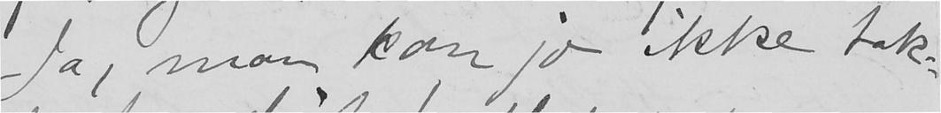Ja, man kan jo ikke tak-
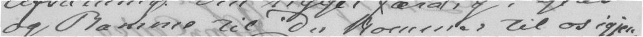og Ramme til Du kommer til os igjen.
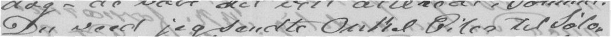Du veed jeg sendte Onkel Eiler til Sølv-
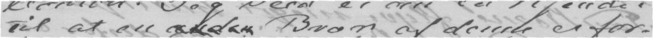til at en anden Bror af denne er for-
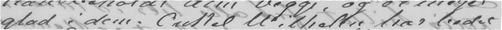glad i dem. Onkel Wilhelm har bedet
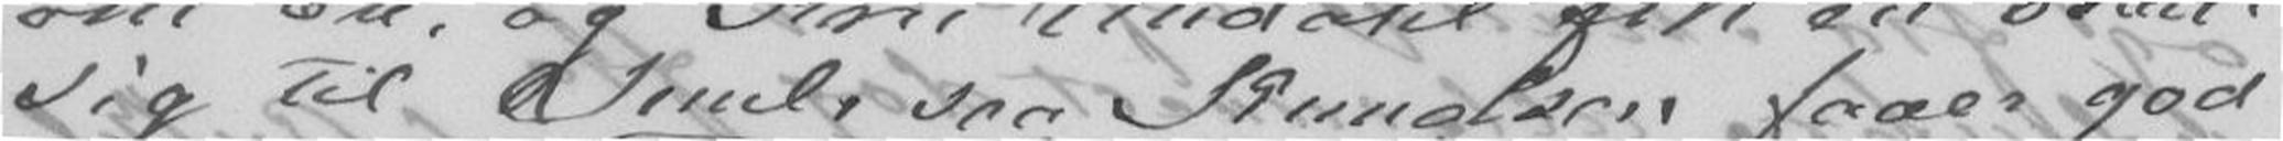sig til Juul, saa Knudsen faaer god
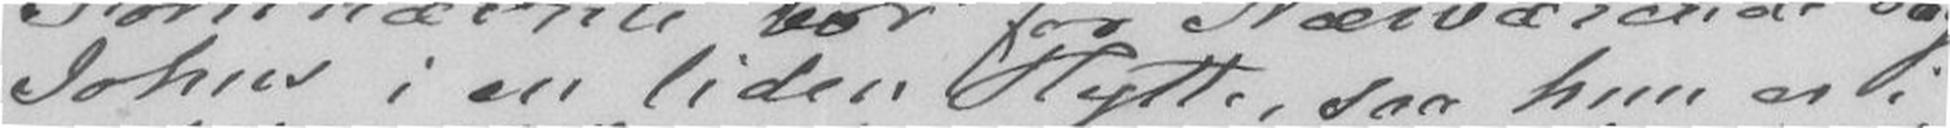John i en liden Hytte, saa hun er i
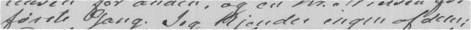første Gang. Jeg kjender ingen af dem;
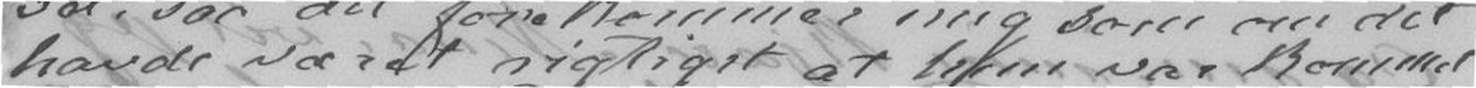havde været rigtigst at hun var kommet
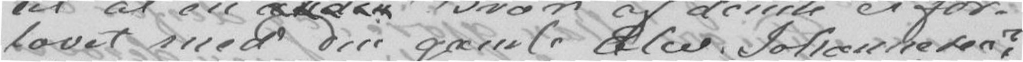lovet med Din gamle Elev. Johannesen?
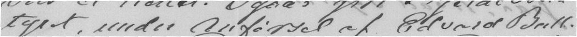tyret, under Anførsel af Edvard Bull.
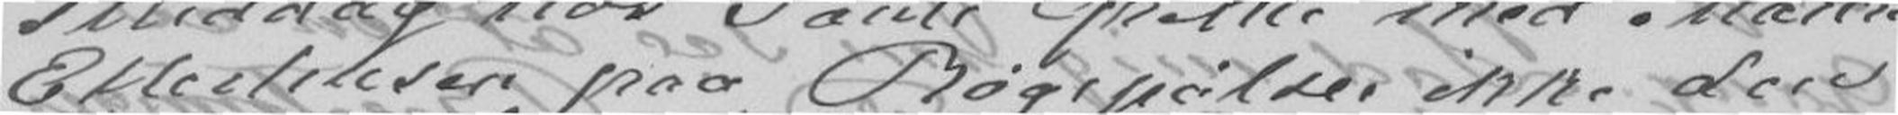Ellerhusen paa Røgepølser ikke den
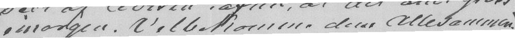imorgen. Velbekomme dem Allesammen
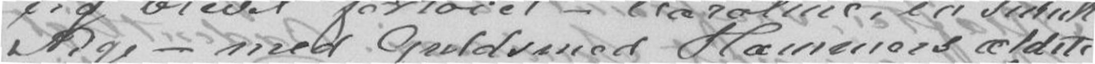Pige - med Guldsmed Hammers ældste
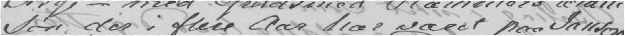Søn, der i flere Aar har varet paa Jansons
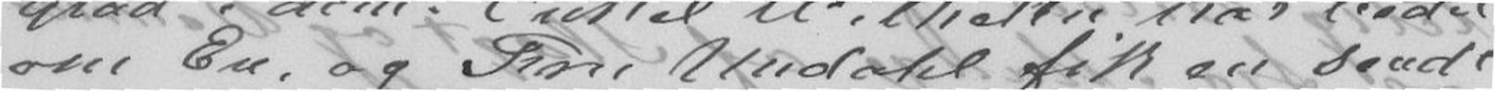om En, og Fru Undahl fik en sendt
